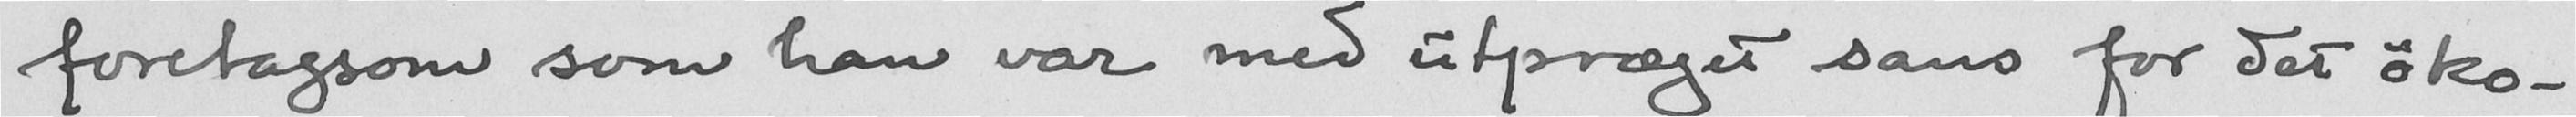foretagsom som han var med utpræget sans for det øko-
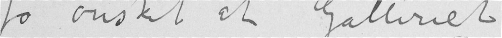jo ønsket at Galleriet
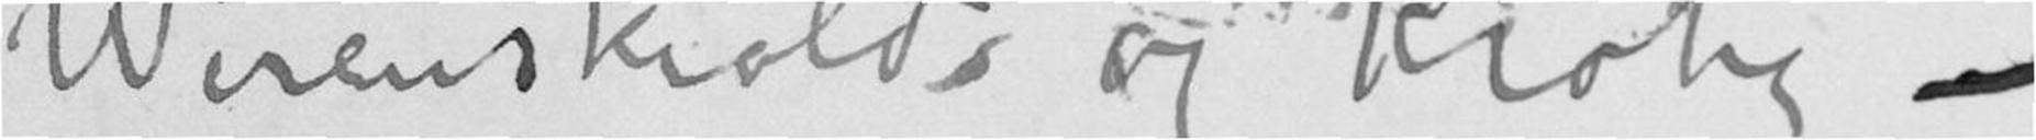Werenskiold og Krohg –
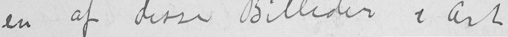en af disse Billeder i Art
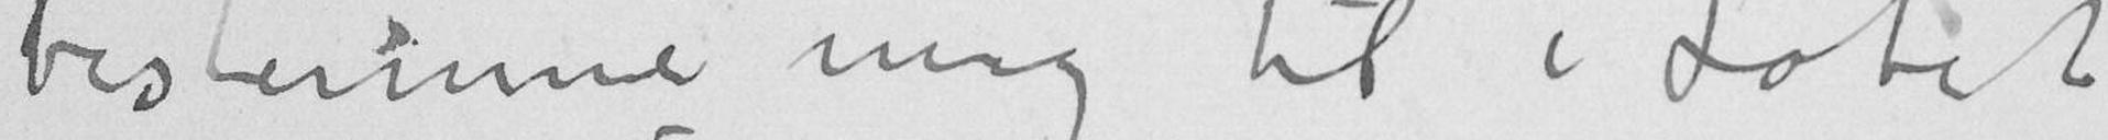bestemme mig til i Løbet
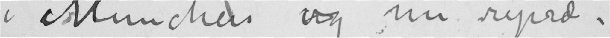i München og nu repræ-
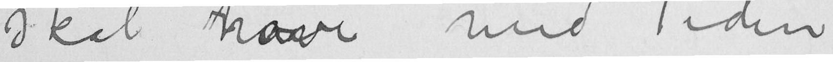skal have med Tiden
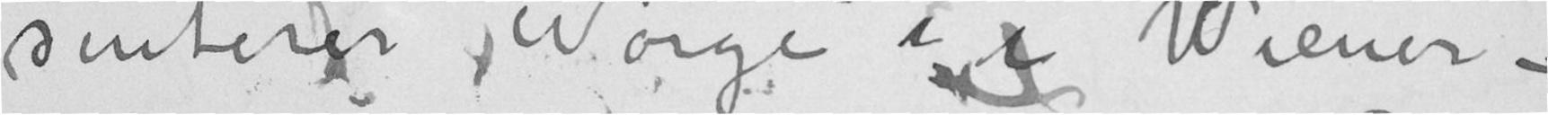senterer Norge i Wiener-
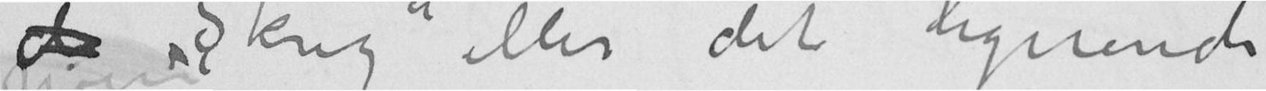hd «Skrig» eller det lignende
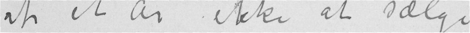af et År ikke at sælge
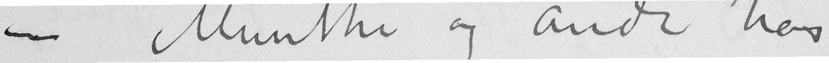– Munthe og Andre har
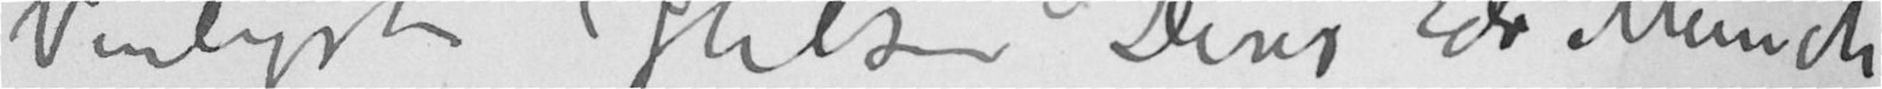Venligst Hilsen Deres Edv. Munch
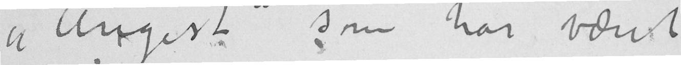«Angest» som har været
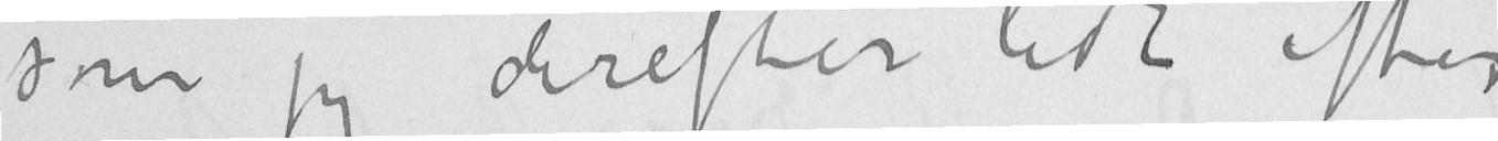som jeg derefter lidt efter
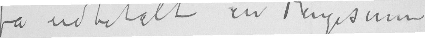få udbetalt en Pengesum
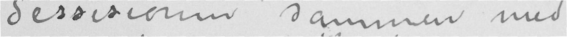Sessesionen sammen med
